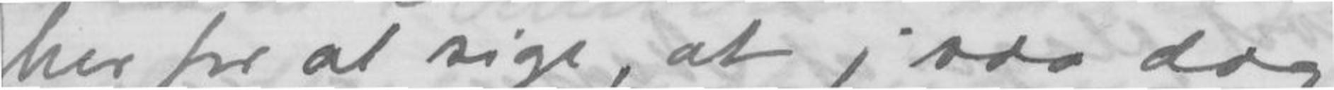her for at sige, at j saa dog
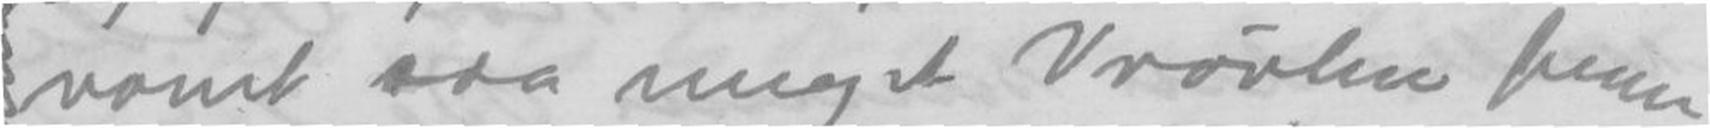vaeret saa meget Vrøvlere frem
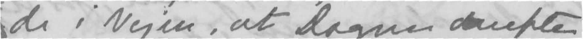de i Vejen, at Dagene derefter
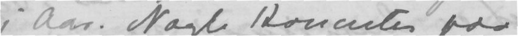i Aar. Nogle Koncerter paa
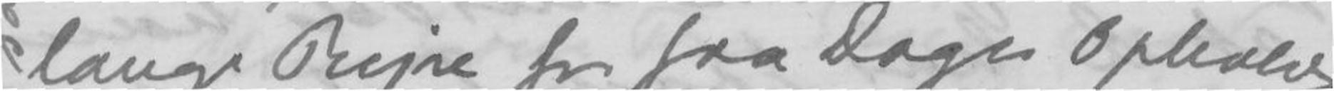lange Rejse for faa Dager Opholds
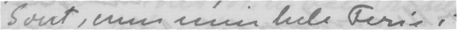Gaest, men min hele Ferie i
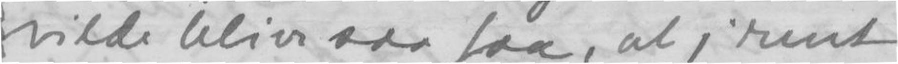vilde blive saa faa, at j rent
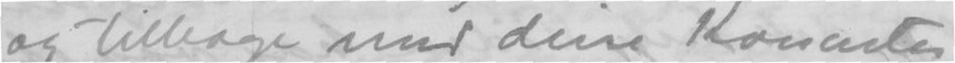og tilbage med dine Koncerter.
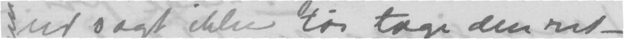ud sagt ikke tør tage den ret-
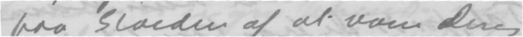paa Glaeder af at vaere Deres
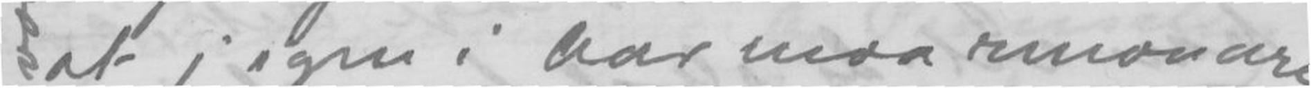at j igen i Aar maa renoncere
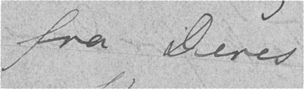fra Deres
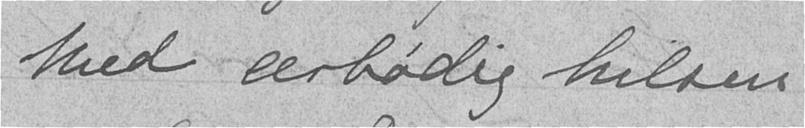Med ærbødig hilsen
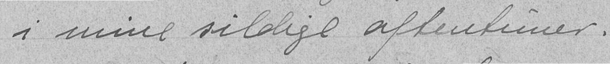i mine sildige aftentimer.
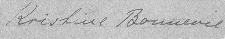Kristine Bonnevie
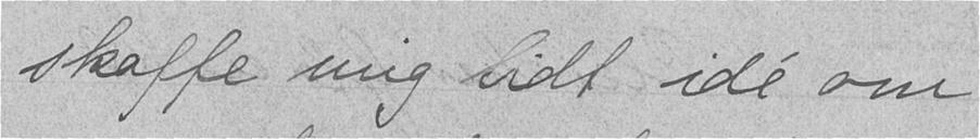skaffe mig lidt ide om
